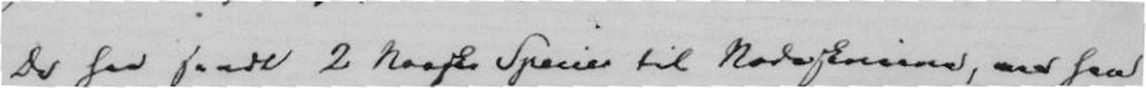Da saa sandt 2 Norske Speciel til Nodaskvinnen, med saa
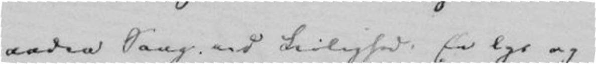saadan Sang ved Leilighed. En lys og
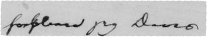Forbliver jeg Deres
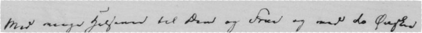Med megen hilsener til Dem og frue og med de Ønsker
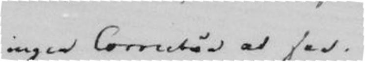ingen Correctød at faa.
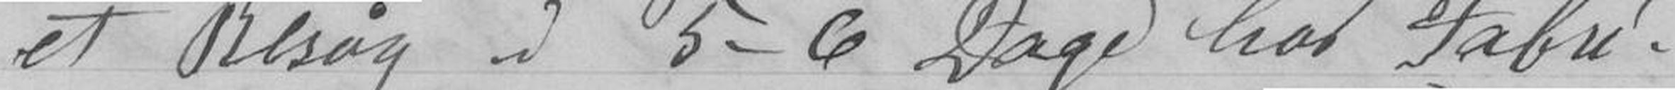et Besøg i 5-6 Dage hos Fabri-
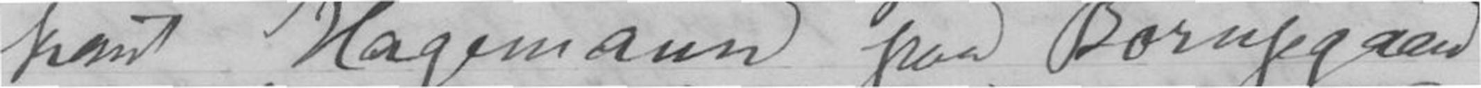kant Hagemann paa Borupgaard
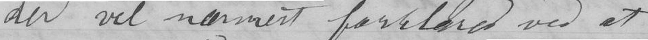der vel nærmest forklares ved at
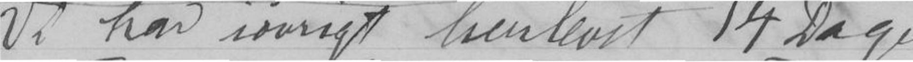Vi har iøvrigt henlevet 14 Dage
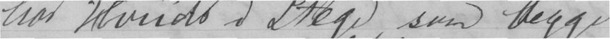hos Hviids i Stege, som begge
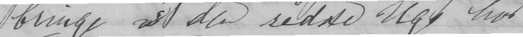bringe vi den sidste Uge hos
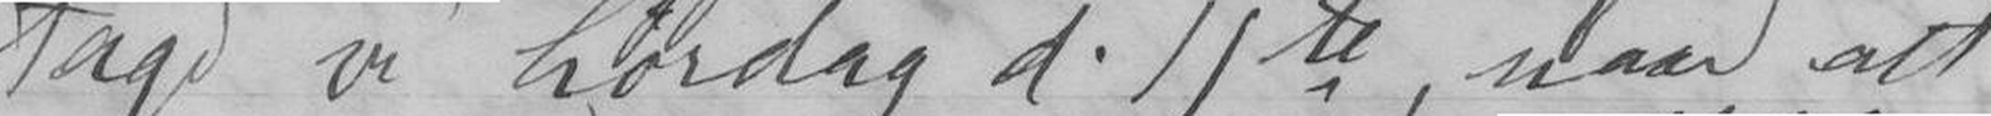tage vi Lørdag d. 11te, naar alt
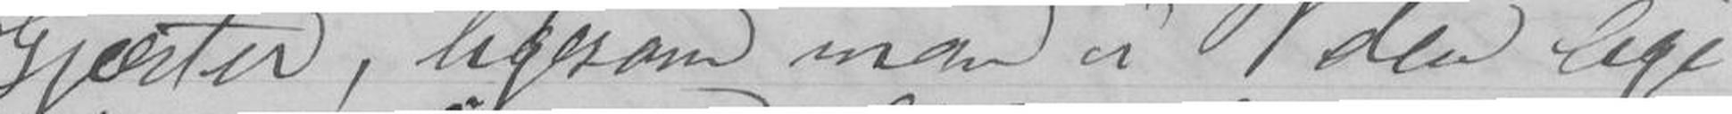Gjæster, ligesom man i den lige
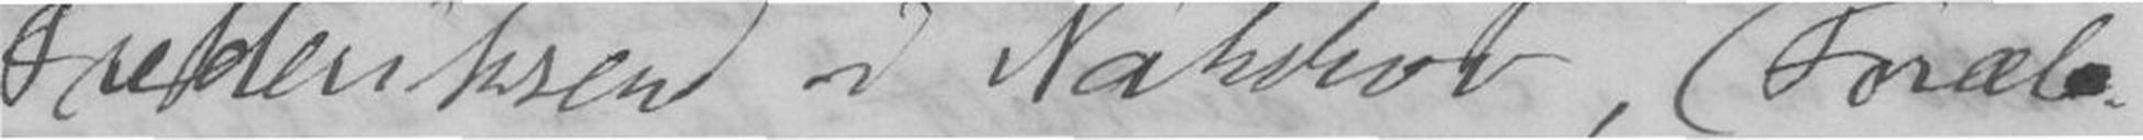Frederiksen i Nahshov, (Foræl
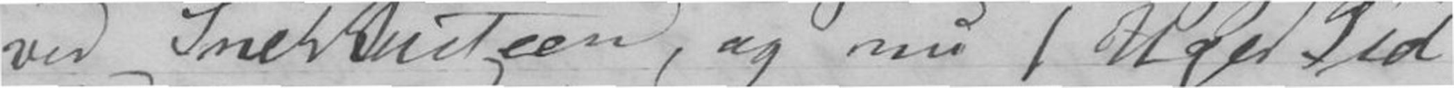ved Snekkersteen, og nu 1 Uges Tid
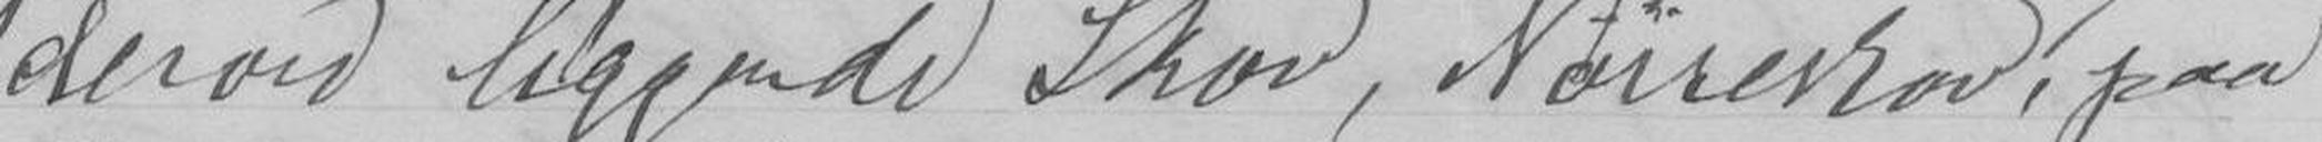derved liggende Skov, Nørreskov, paa
